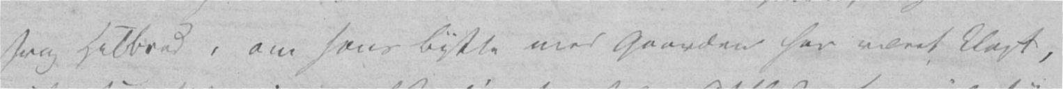svag Helbred, om hans Bytte med Gaarden har været klogt,
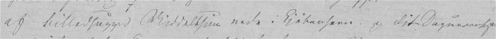af Billedhugger Middelthun nede i Kjøbenhavn. og Dit Daguerroty-
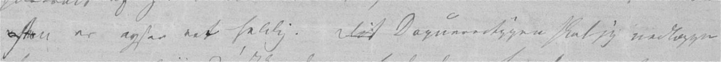sten er ogsaa ret heldig. Dit Daguerrotypen skal jeg nedlægge
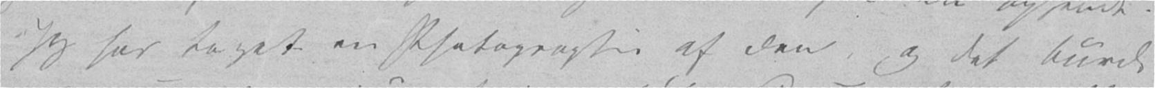Jeg har taget en Photographie af den, og det burde
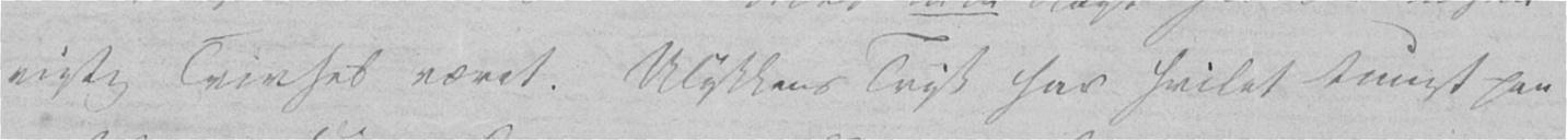rigtig Trivsel været. Ulykkens Tryk har hvilet tungt paa
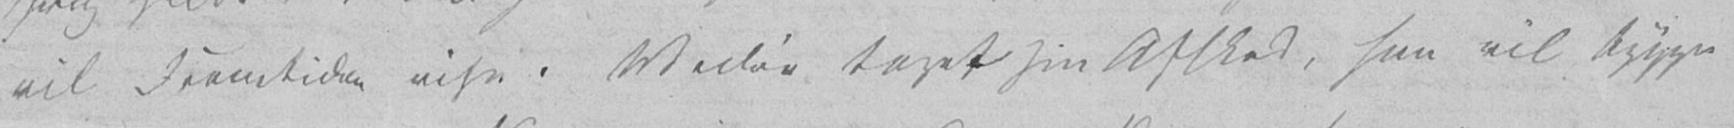vil Fremtiden vise. Vedøe taget sin Afsked, han vil bygge
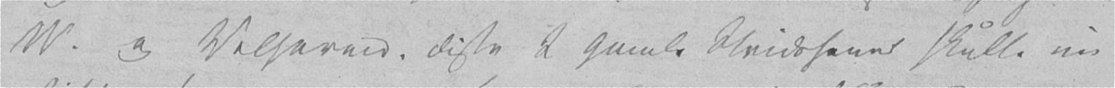W. og Welhaven, disse 2 gamle Stridshaner skulle nu
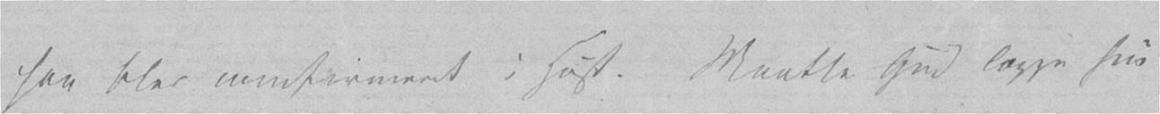han blev comfirmeret i Høst. Maatte Gud lægge sin
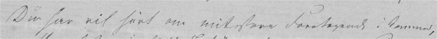Du har vil hørt om mit store Foretagende i Sommer,
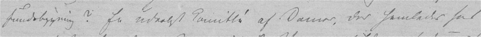Samfundsbygning? En udvalgt Komitté af Damer, der samledes hos
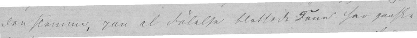den slemme, paa al Følelse blottede Kone har ganske
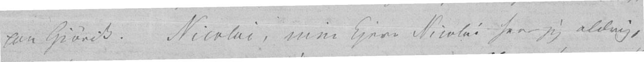paa Gjøvik. Nicolai, min kjere Nicolai seer jeg aldrig,
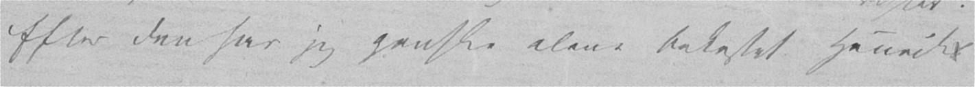Efter den har jeg ganske alene bekostet Henriks
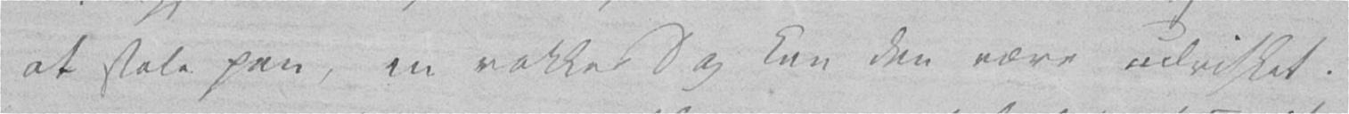at stole paa, en vakker Dag kan den være udvisket.
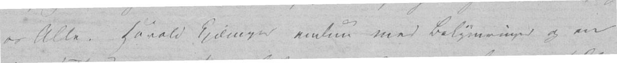os Alle. Harald kjæmper endnu med Bekymringer og en
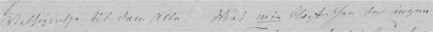Velsignelse til dem Alle. Med min Slægt har der ingen
